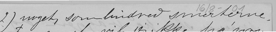2) noget, som lindred smærterne.
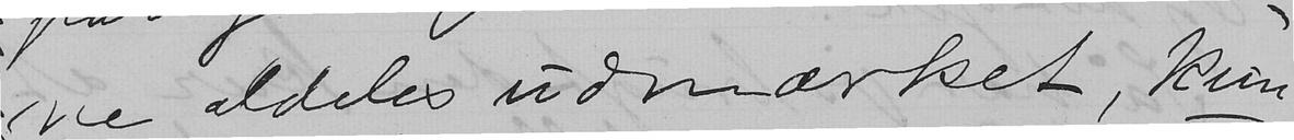ne aldeles udmærket, kun
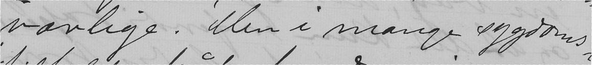værlige. Men i mange sygdoms-
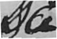så
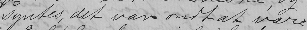syntes, det var ondt at være
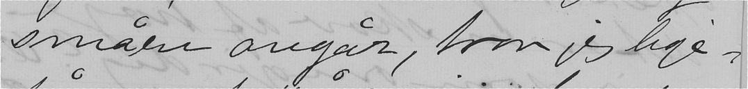småen angår, tror jeg lige-
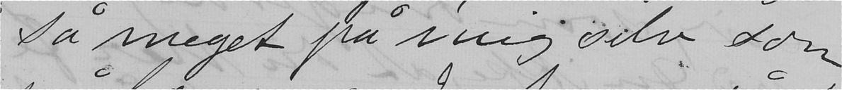så meget på mig selv som
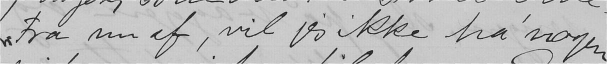Fra nu af, vil jeg ikke ha' nogen
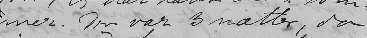mer. Der var 3 nætter, da
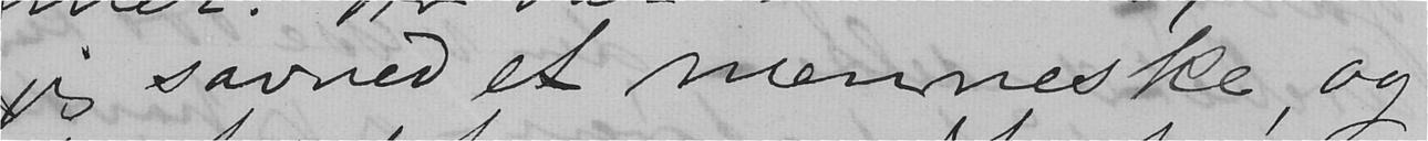jeg savned et menneske, og
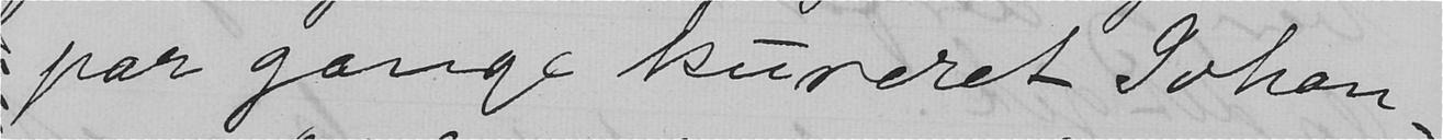par gange kureret Johan-
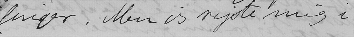linger. Men jeg rejste mig i
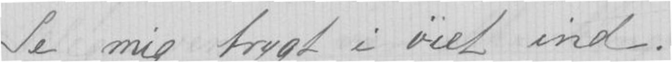Se mig trygt i øiet ind.
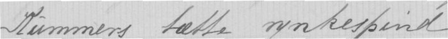Kummers tætte rynkespind
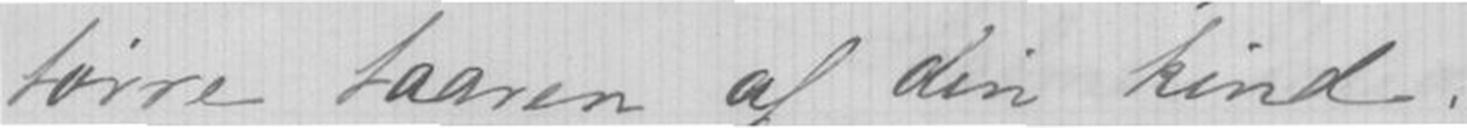tørre taaren af din kind.
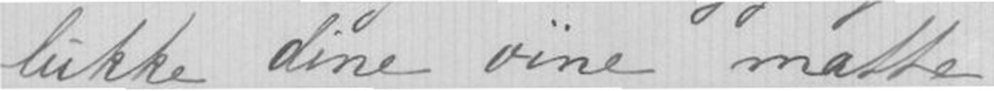lukke dine øine matte,
